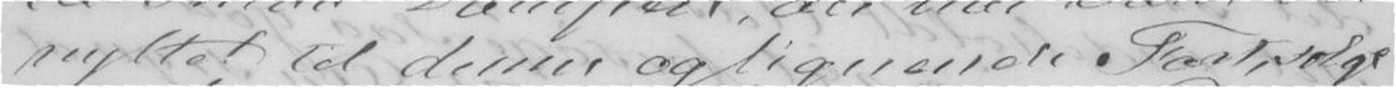nyttet til denne og lignende Fart, solgt
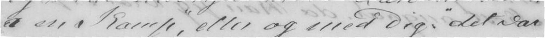en Kamp", eller og med Dig: "det var
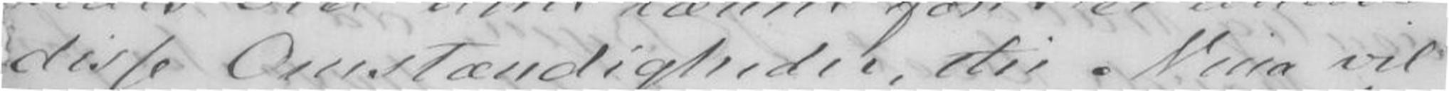disse Omstændigheder, thi Nina vil
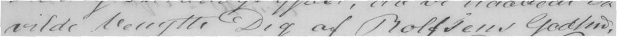vilde benytte Dig af Rolfsens Godhed,
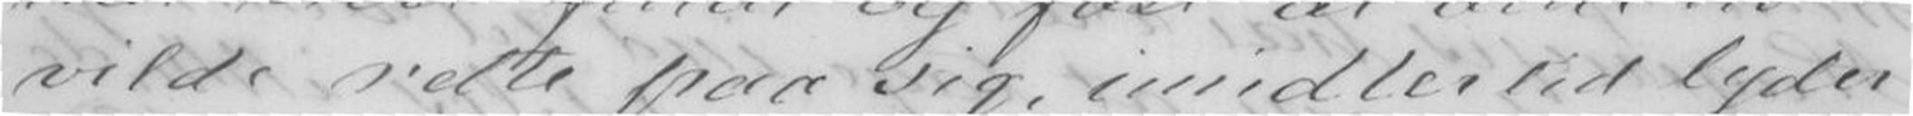vilde rette paa sig, imidlertid lyder
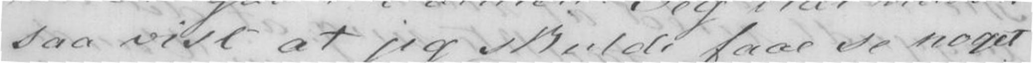saa vist at jeg skulde faae se noget
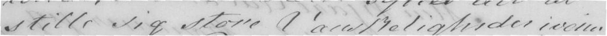stille sig store Vanskeligheder iveien
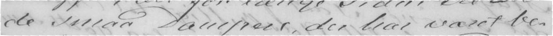de smaa Dampere, der har været be-
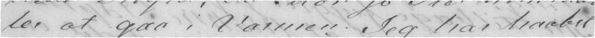ler at gaa i Varmen. Jeg har haabet
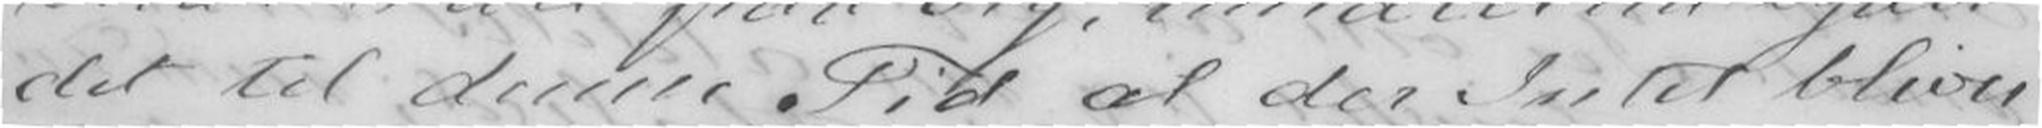det til denne Tid at der Intet bliver
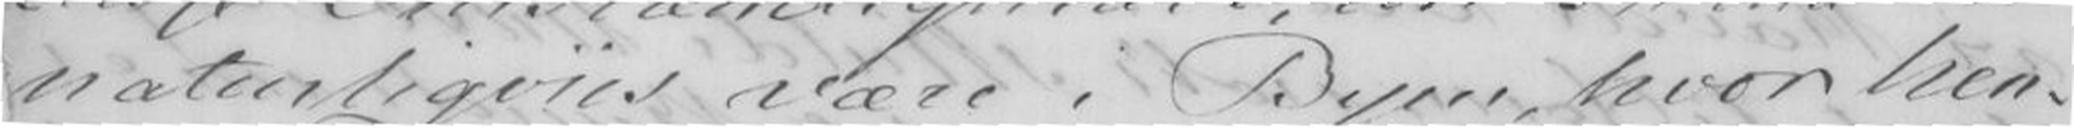naturligviis være i Byen, hvor hen-
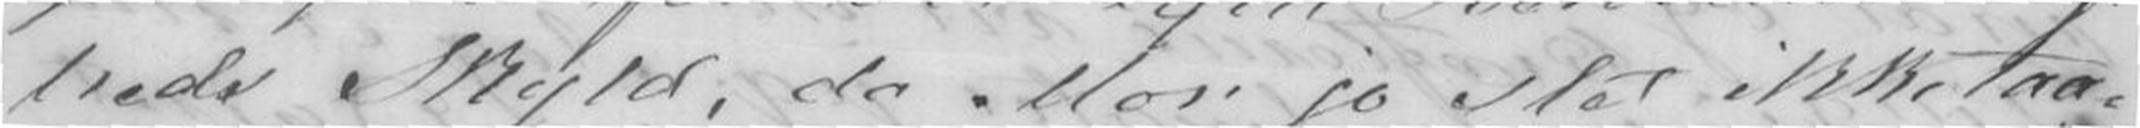heds Skyld, da Mor jo slet ikke taa-
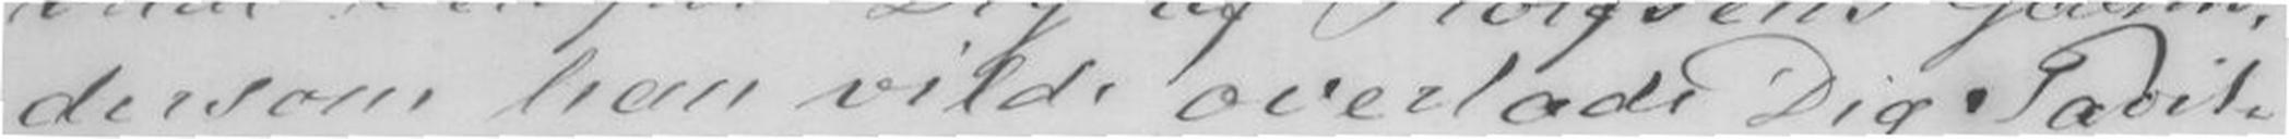dersom han vilde overlade Dig Pavil-
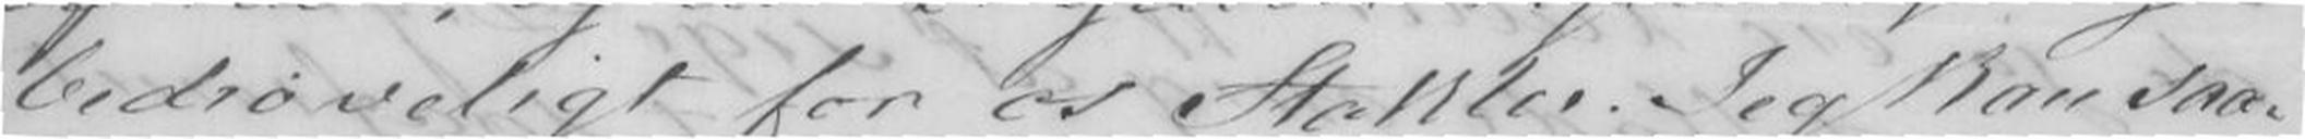bedrøveligt for os Stakler. Jeg kan saa-
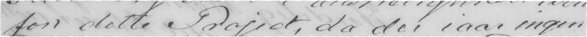for dette Project, da der iaar ingen
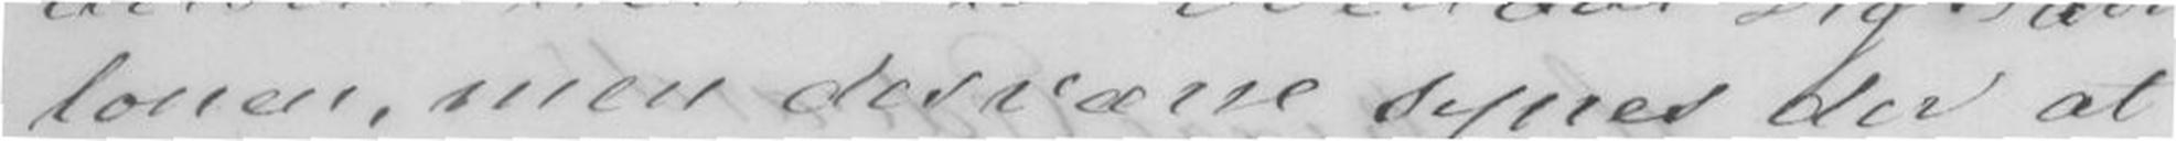lonen, men desværre synes der at
